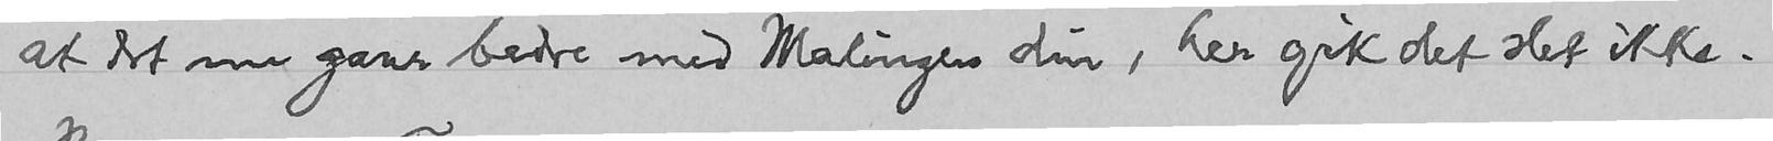at det nu gaar bedre med Malingen din, her gik det slet ikke.
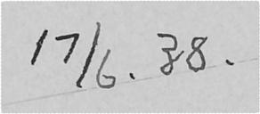17/6. 38.
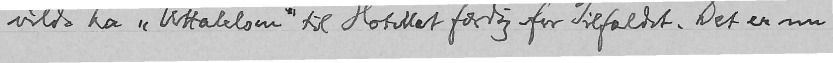vilde ha "Uttalelsen" til Hotellet færdig for Tilfældet. Det er nu
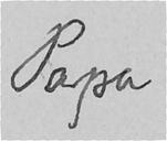Papa
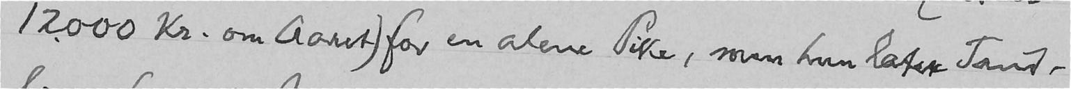12.000 Kr. om Aaret) for en alene Pike, men hun later Tand-
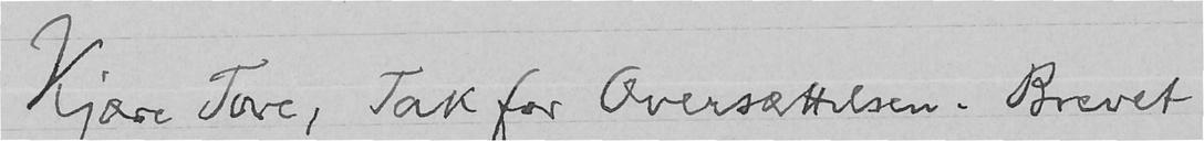Kjære Tore, Tak for Oversættelsen. Brevet
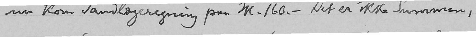nu kom Tandlægeregning paa M. 160. - Det er ikke Summen,
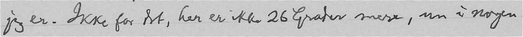jeg er. Ikke for det, her er ikke 26 Grader mere, nu i nogen
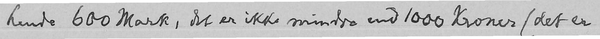hende 600 Mark, det er ikke mindre end 1000 Kroner (det er
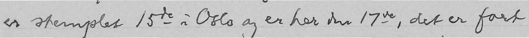er stemplet 15de i Oslo og er her den 17de, det er fort
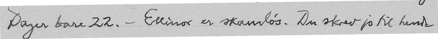Dager bare 22. - Ellinor er skamløs. Du skrev jo til hende
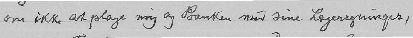om ikke at plage mig og Banken med sine Lægeregninger,
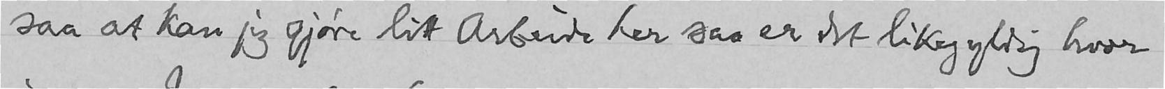saa at kan jeg gjøre litt Arbeide her saa er det likegyldig hvor
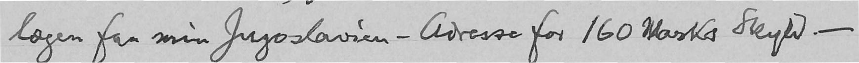lægen faa min Jugoslavien-Adresse for 160 Marks Skyld -
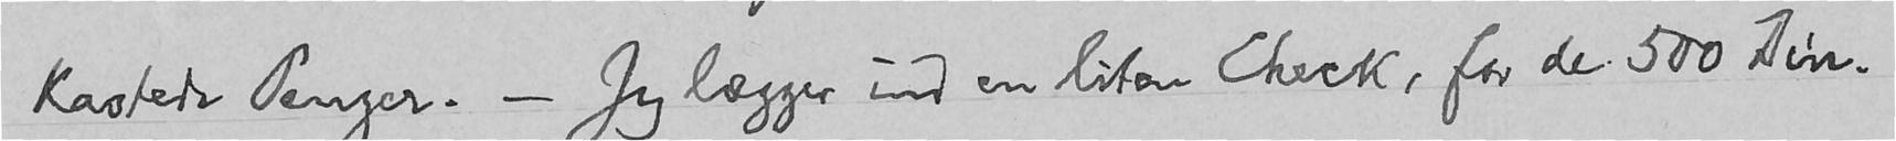kastede Penger. - Jeg lægger ind en liten Check, for de 500 Din.
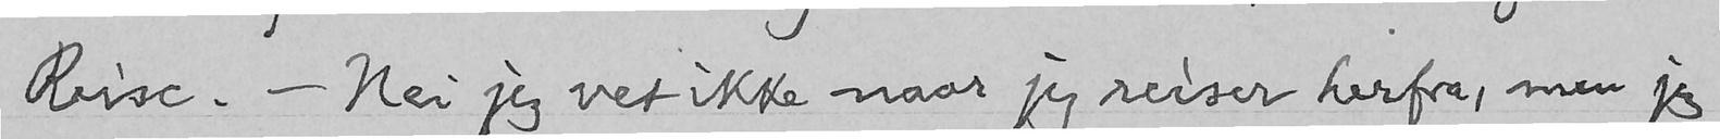Reise. - Nei jeg vet ikke naar jeg reiser herfra, men jeg
